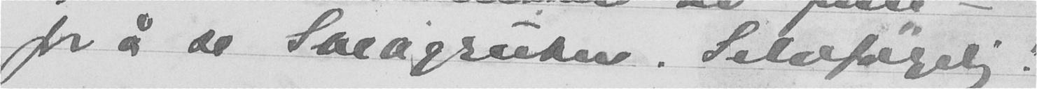for å se Sveagruben. Selvfølgelig!
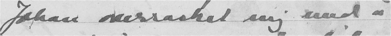Johan overrasket mig med å
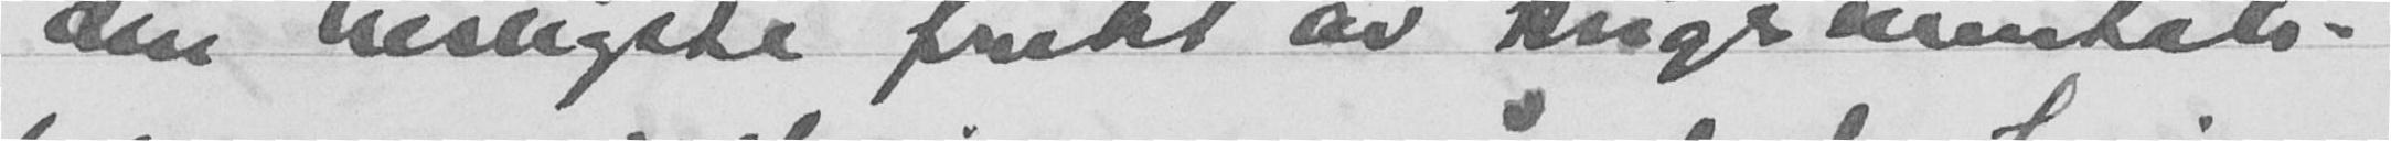den hesligste frukt av krigsmentali-
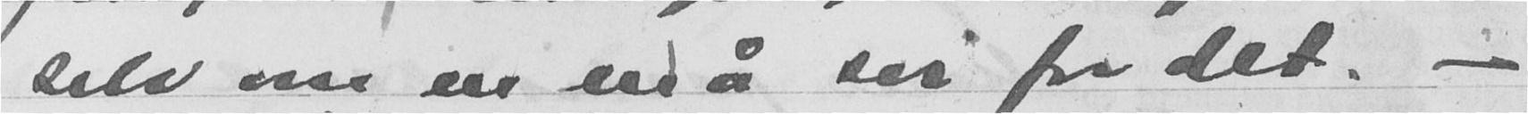selv om en må svi for det. -
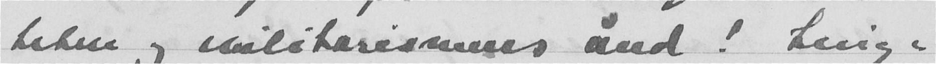teten og militarismens ånd! Krigs-
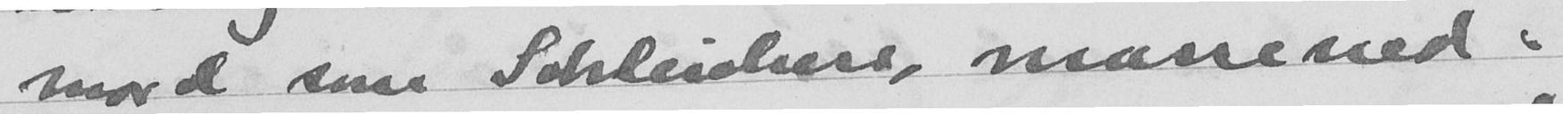mord som Schleichuess, massenød,
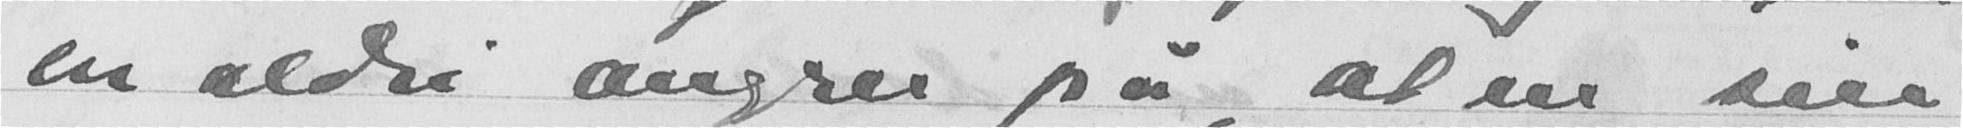en aldri angrer på, at en sier
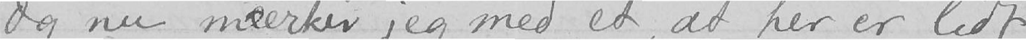Og nu mærker jeg med et, at her er lidt
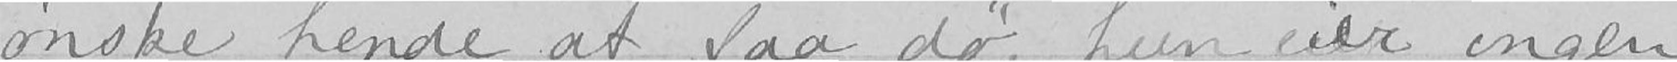ønske hende at faa dø, hun eier ingen
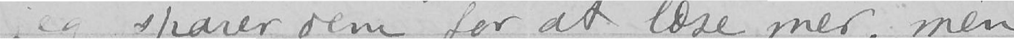jeg sparer dem for at læse mer, men
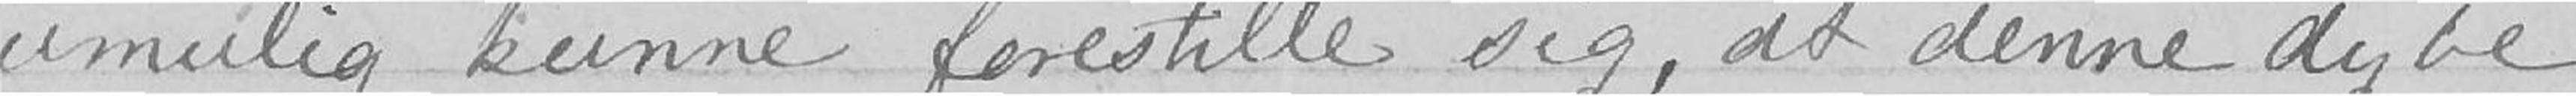umulig kunne forestille sig, at denne dybe
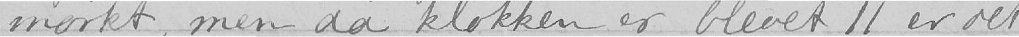mørkt, men da klokken er blevet 11 er det
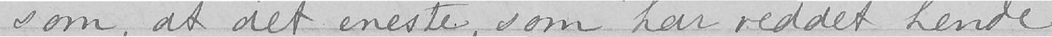som, at det eneste, som har reddet hende
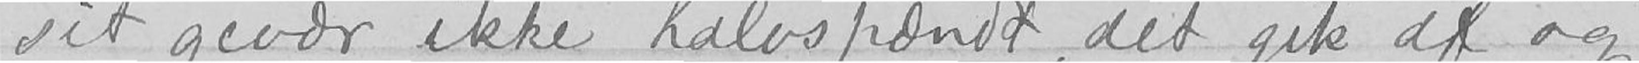sit gevær ikke halvspændt, det gik af og
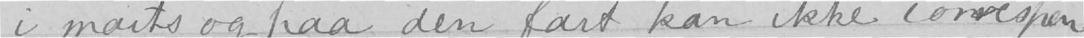i marts og paa den fart kan ikke correspon-
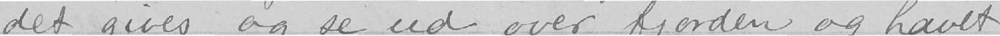det gives, og se ud over fjorden og havet,
